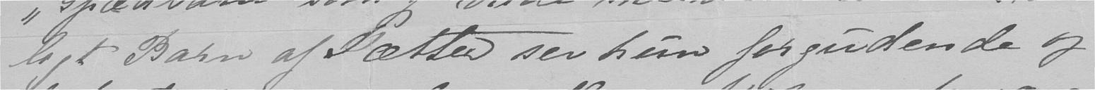ligt Barn af Sætter ser hun forgudende og
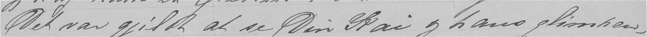Det var gjildt at se Din Kai og hans glimren-
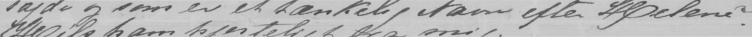sagde og som er et tænkelig Navn efter Helene?
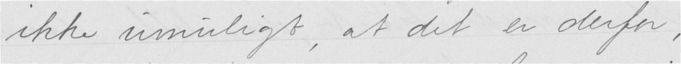ikke umuligt, at det er derfor,
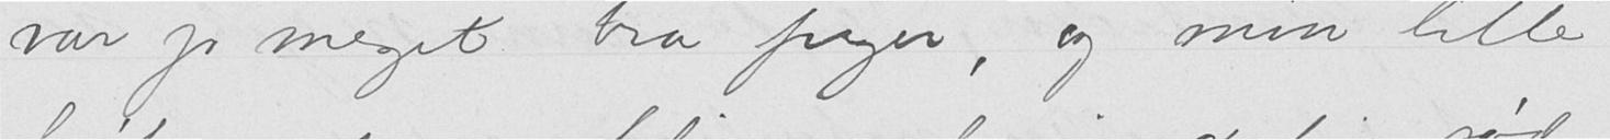var jo meget bra piger, og min lille
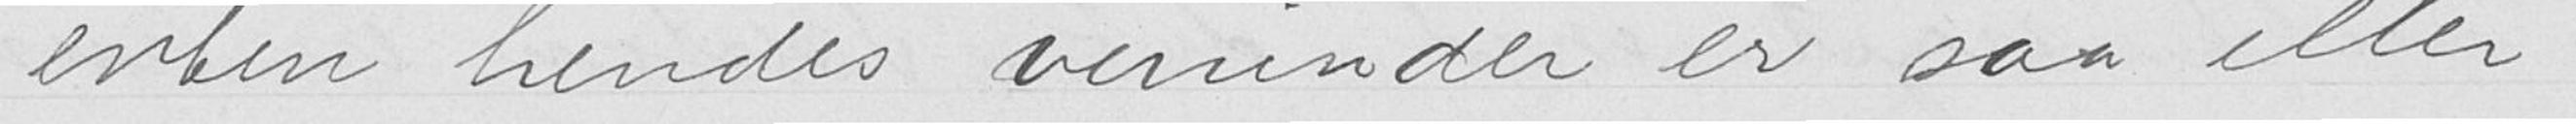enten hendes veninder er saa eller
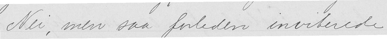Nei, men saa forleden inviterede
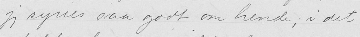jeg synes saa godt om hende; i det
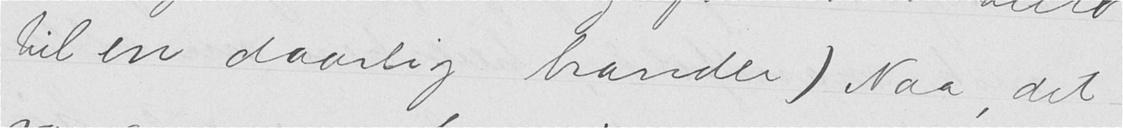til en daarlig brander) Naa, det
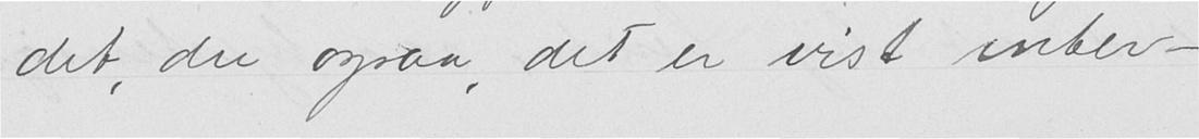det, du ogsaa, det er vist inter-
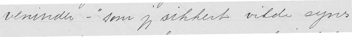veninder - "som jeg sikkert vilde synes
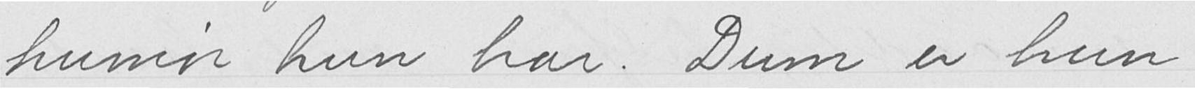humør hun har. Dum er hun,
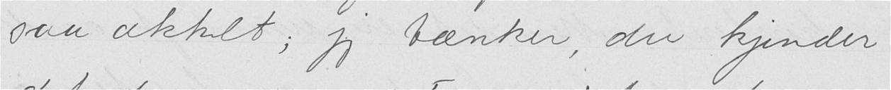saa ækkelt; jeg tænker, du kjender
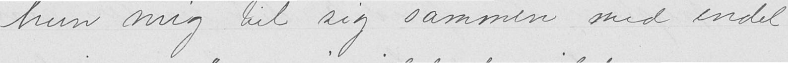hun mig til sig sammen med endel
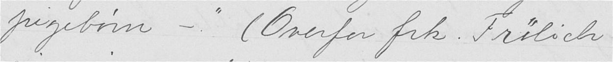pigebørn - " (Overfor frk. Frølich
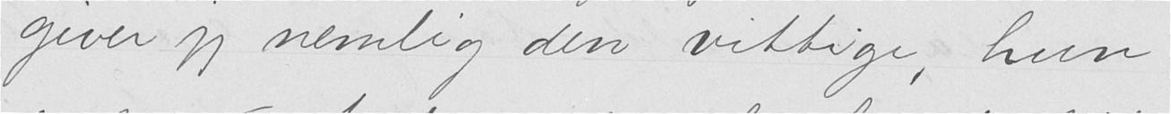giver jeg nemlig den vittige, hun
